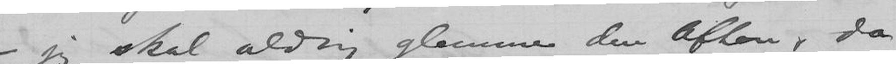- jeg skal aldrig glemme den Aften, da
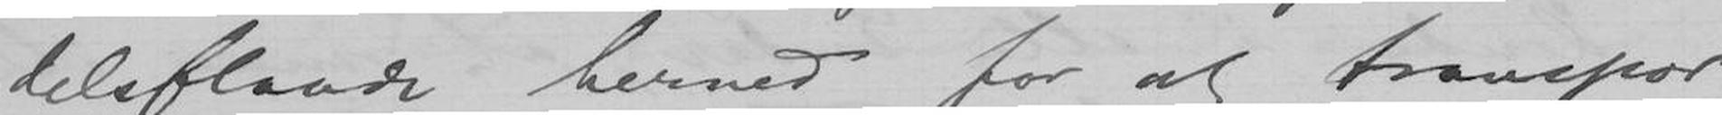delsflaade herned for at transpor-
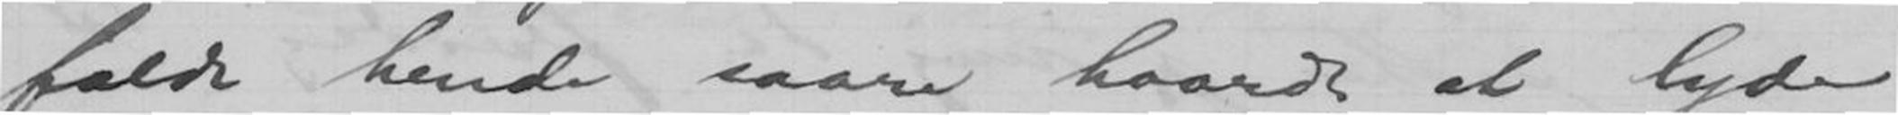falde hende saare haardt at lyde
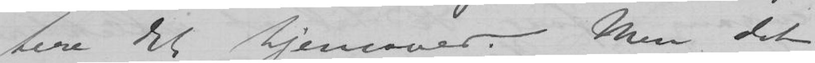tere det hjemover. Men det
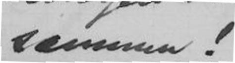sammen!
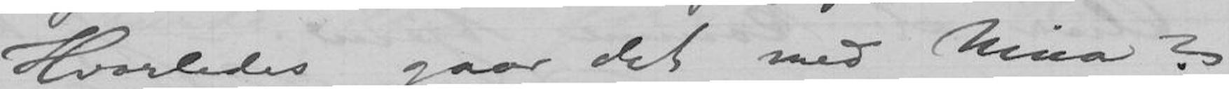Hvorledes gaar det med Nina?
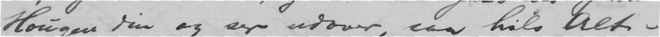Hougen din og ser udover, saa hils Alt-
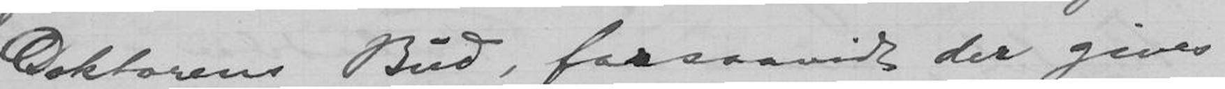Doktorens Bud, forsaavidt der gives
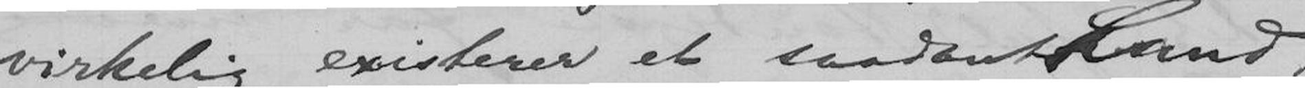virkelig existerer et saadant Land,
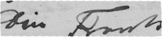Din Frants.
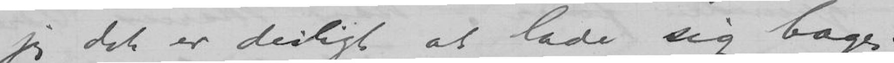jeg det er deiligt at lade sig bage.
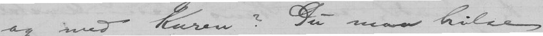og med Kuren? Du maa hilse
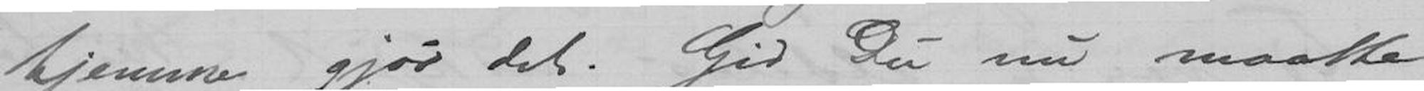hjemme gjør det. Gid Du nu maatte
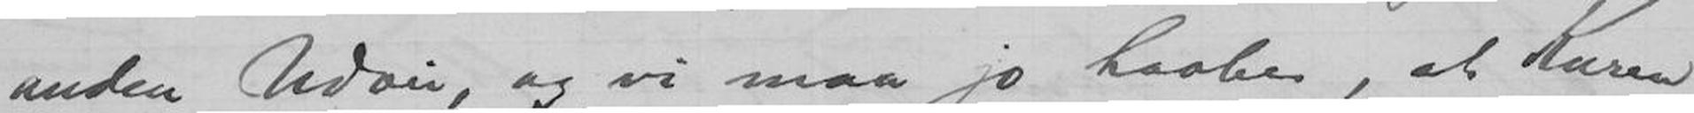anden Udvei, og vi maa jo haabe, at Kuren
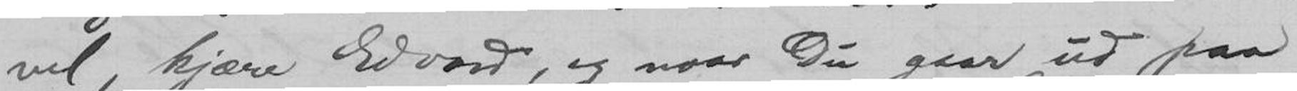vel, kjære Edvard, og naar Du gaar ud paa
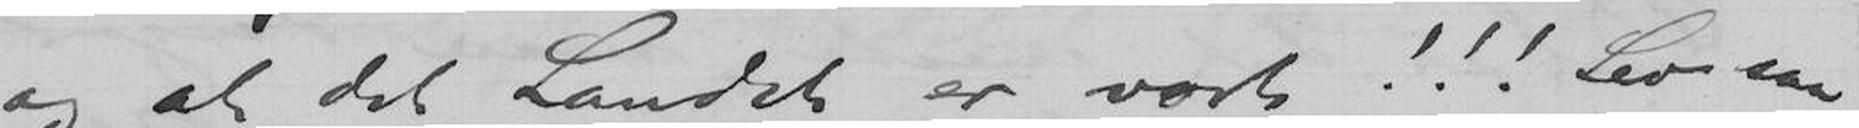og at det Landet er vort!!! Lev saa
